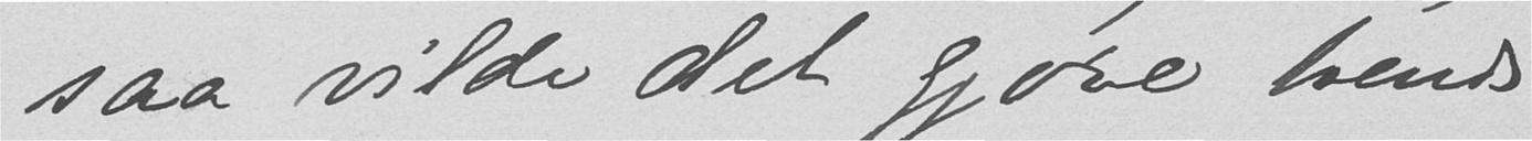saa vilde det gjøre hende
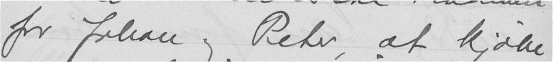for Johan og Peter, at kjøbe
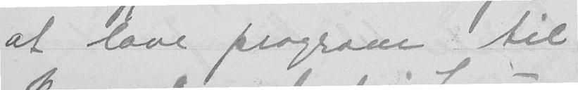at lave program til
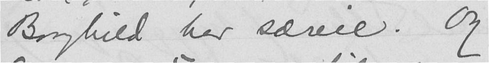Borghild har særeie. Og
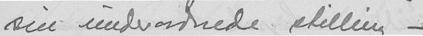sin underordnede stilling -
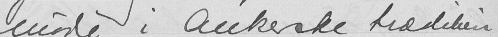møde i Ankerske træsliberi
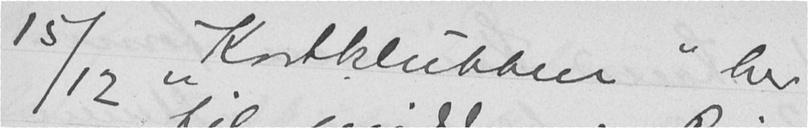15/12 "Kortklubben" her
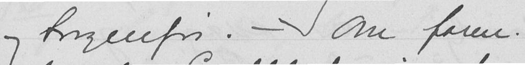og Sorgenfri. - Om form.
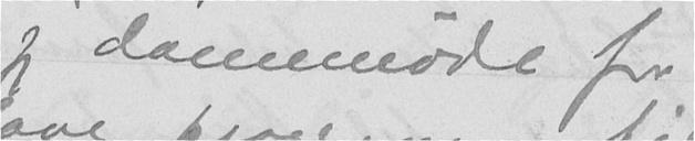jeg damemøde for
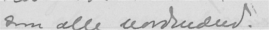som alle nordmænd.
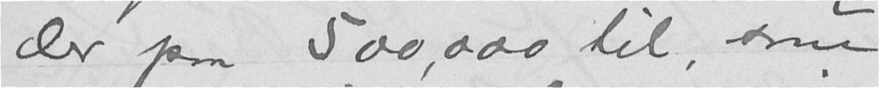der paa 500,000 til, som
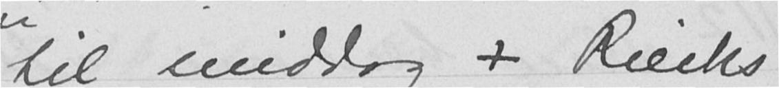til middag + Riecks
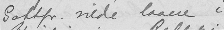Gottfr. vilde laane i
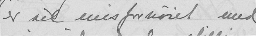er vel misfornøiet med
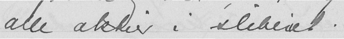alle aktier i sliberet.
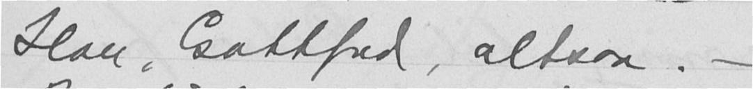Han, Gottfred, altsaa. -
